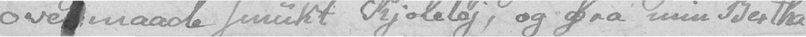ovemaade smukt Kjoletøj, og fra min Bertha
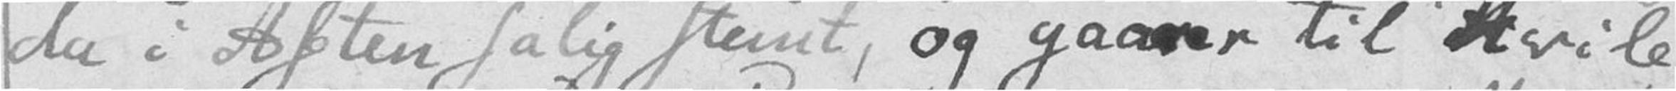da i Aften salig stemt, og gaaer til Hvile
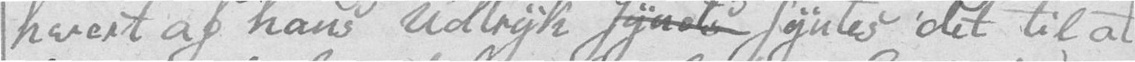hvert af hans Udtryk synets syntes det til at
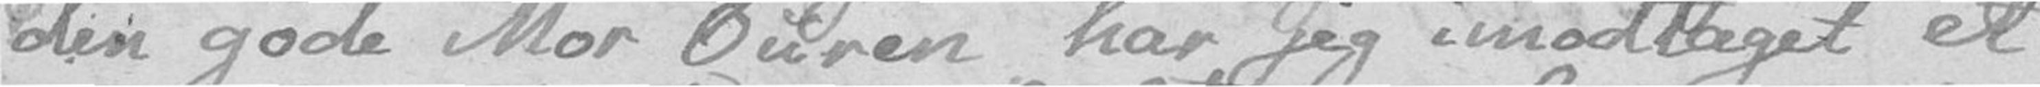den gode Mor Ouren har jeg imodtaget et
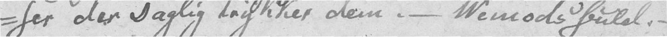-ser der Daglig trykker dem. –  Vemodsfuld. –
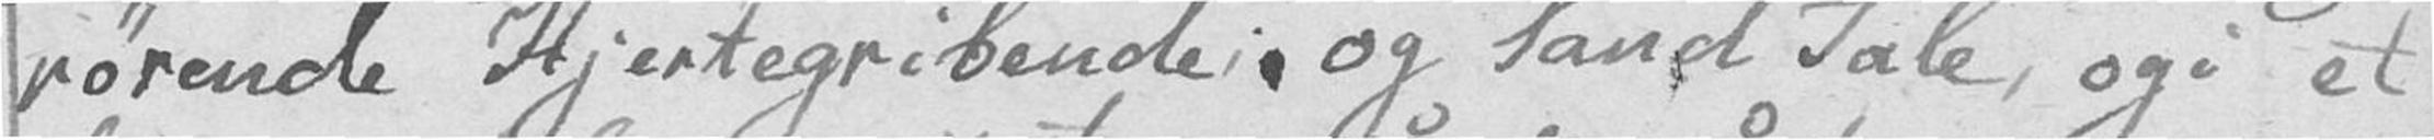rørende Hjertegribende, og Sand Tale, og i et
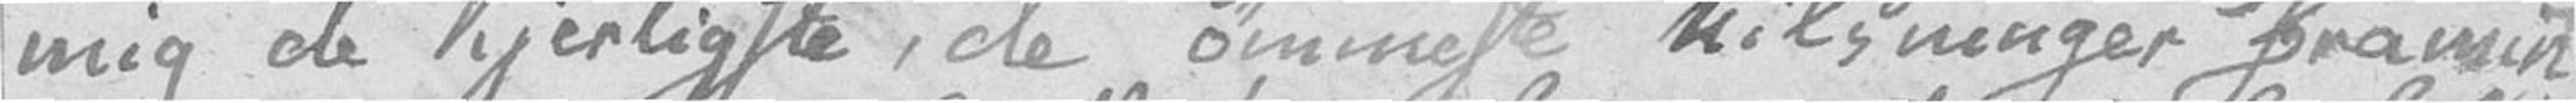mig de kjerligste, de ømmeste hilsninger fra min
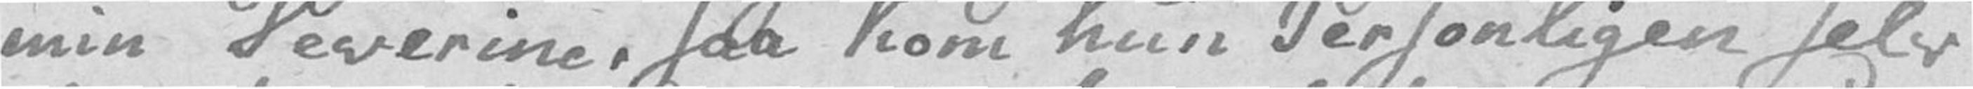min Severine, saa kom hun Personligen selv
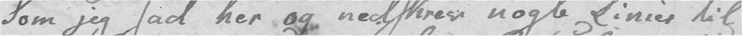Som jeg sad her og nedskrev nogle Linier til
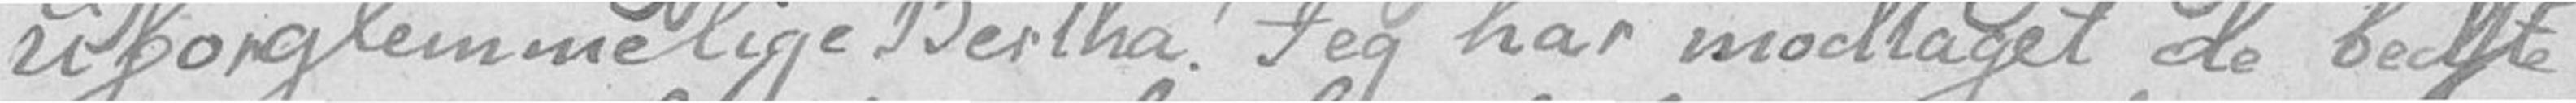Uforglemmelige Bertha! Jeg har modtaget de bedste
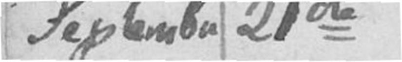September 21de
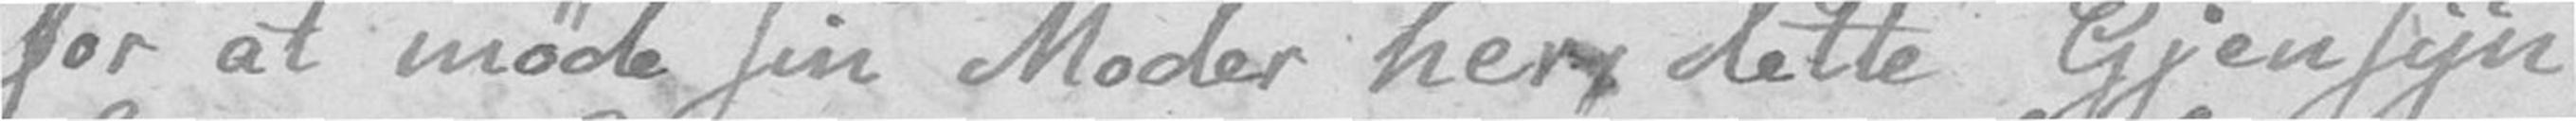for at møde sin Moder her, dette Gjensyn
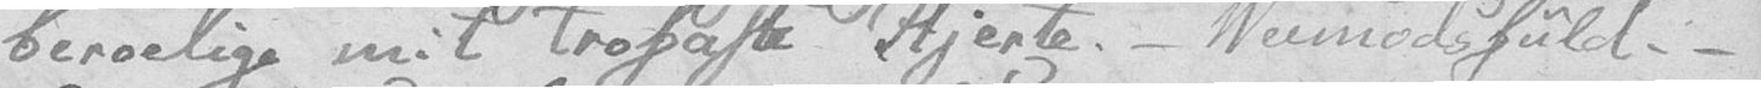beroelige mit trofaste Hjerte. – Veemodsfuld. –
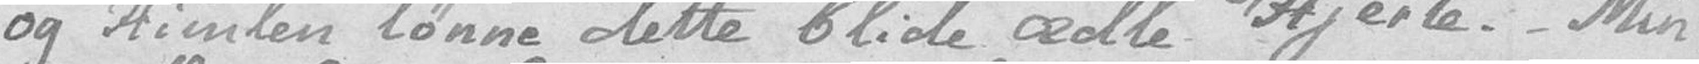og Himlen lønne dette blide ædle Hjerte. – Min
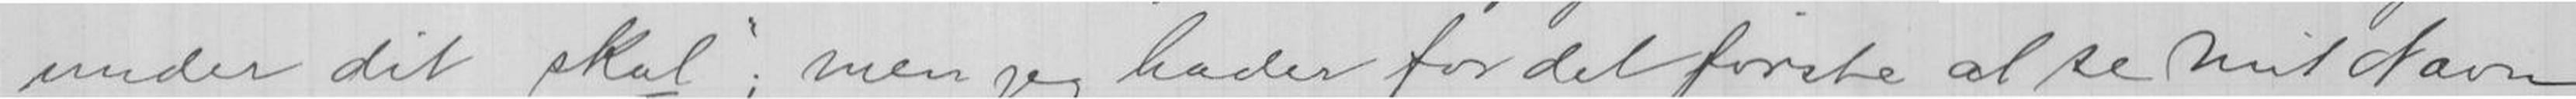under dit skal; men jeg hader for det første at se mit Navn
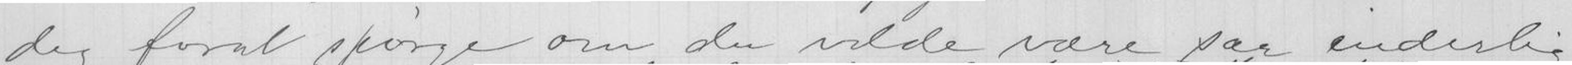dig forat spørge om du vilde være saa inderlig
